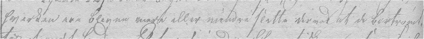hverken ere bleven mere eller mindre slette derved at de bortrømte
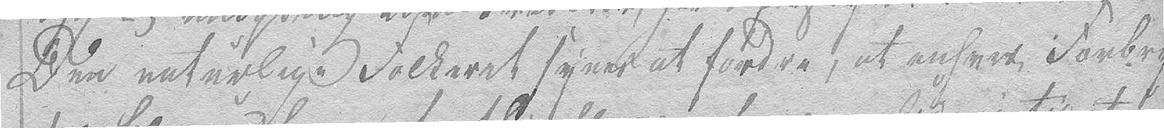Den naturlige Folkeret synes at fordre, at enhver Forbry-
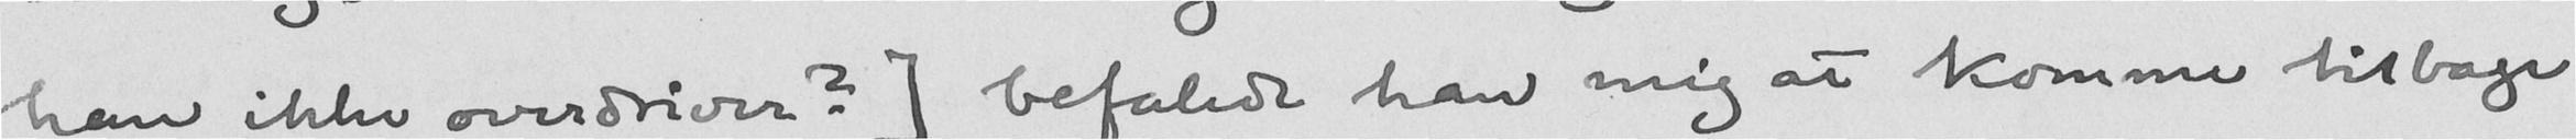han ikke overdriver?) befalede han mig at komme tilbage
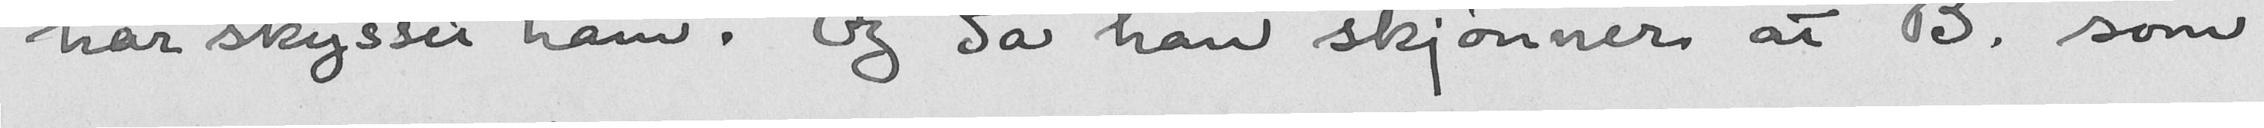har skysset ham. Og da han skjønner at B. som
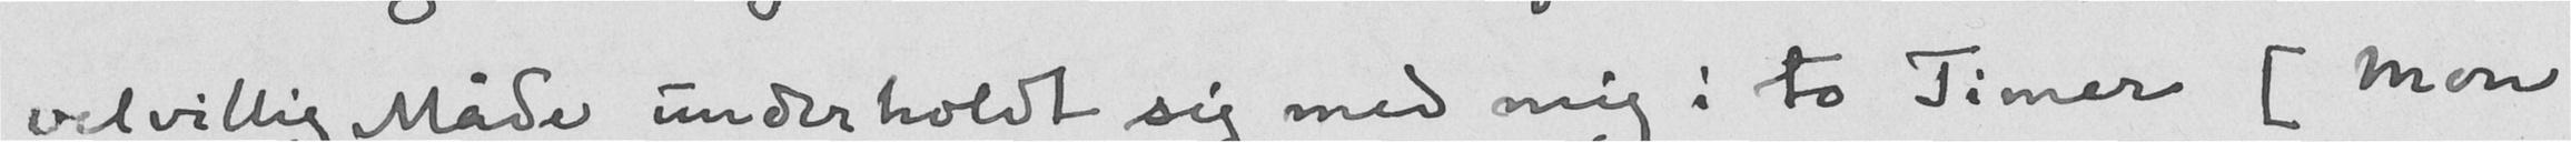velvillig Måde underholdt sig med mig i to Timer (mon
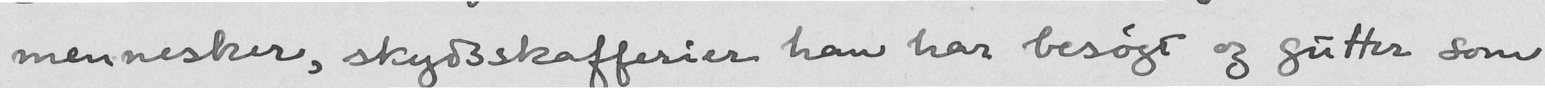mennesker, skydsskafferier han har besøgt og gutter som
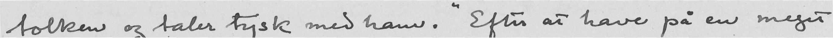tolken og taler tysk med ham. "Efter at have på en meget
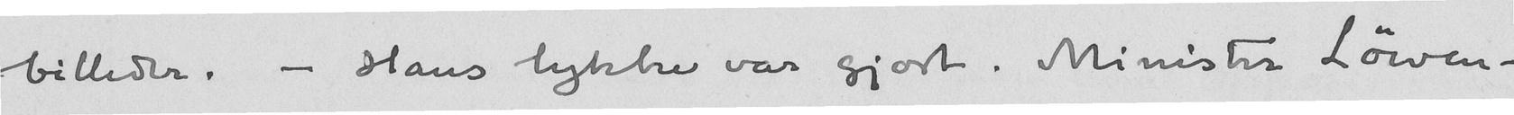billeder. - Hans lykke var gjort. Minister Løwen-
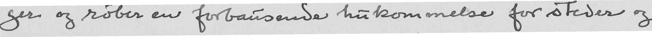ger og røber en forbausende hukommelse for steder og
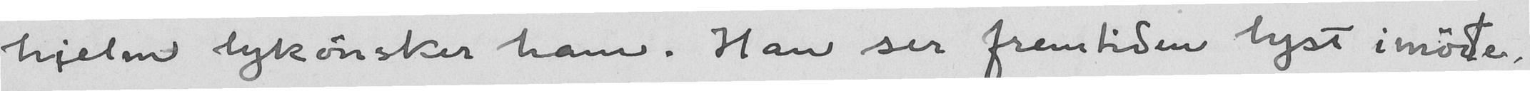hjelm lykønsker ham. Han ser fremtiden lyst imøde.
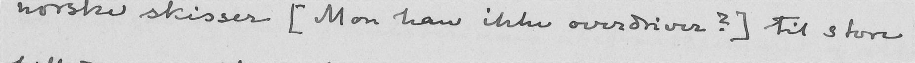norske skisser (Mon han ikke overdriver?) til store
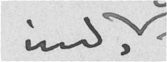ind
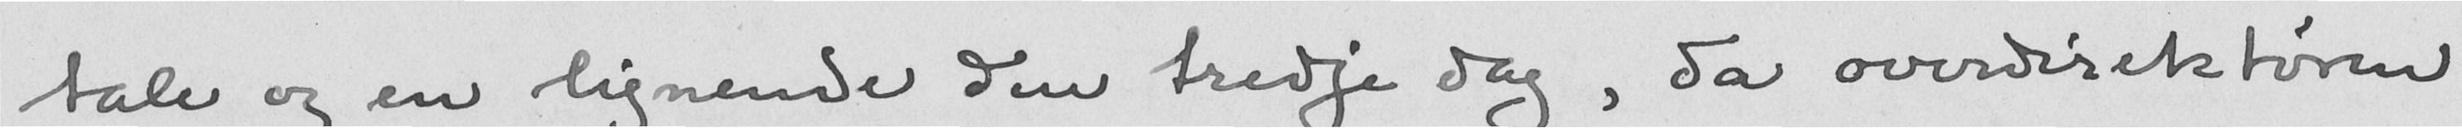tale og en lignende den tredje dag, da overdirektøren
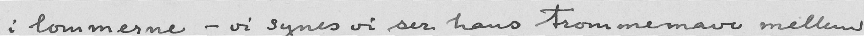i lommerne - vi synes vi ser hans trommemave mellem
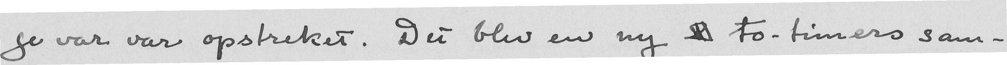ge var var opstreket. Det blev en ny 2 to-timers sam-
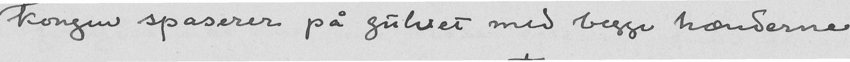kongen spaserer på gulvet med begge hænderne
